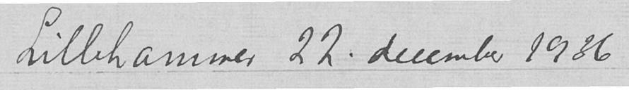Lillehammer 22. december 1936
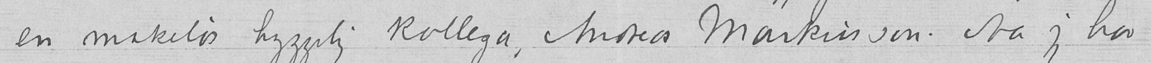en makeløs hyggelig kollega, Andreas Markusson. Aa jeg har
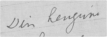Din hengivne
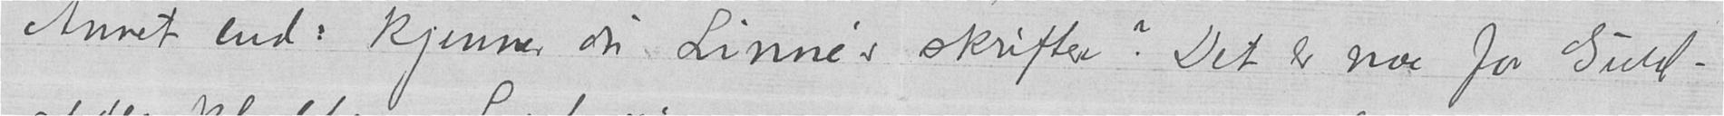Annet end: kjenner du Linnés skrifter? Det er noe for Guld –
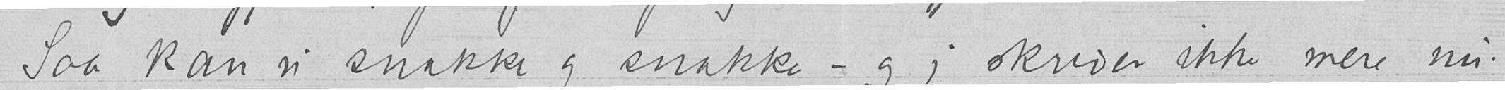Saa kan vi snakke og snakke – og jeg skriver ikke mere nu.
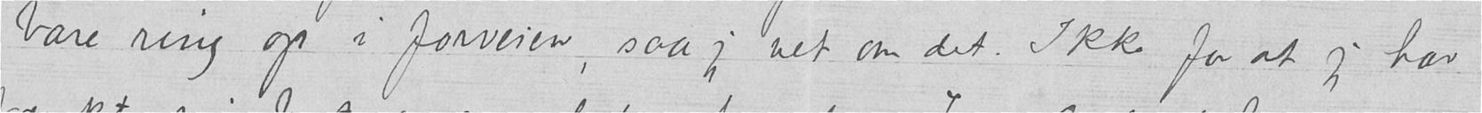- bare ring op i forveien, saa jeg vet om det. Ikke for at jeg har
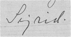Sigrid.
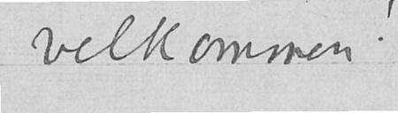velkommen!
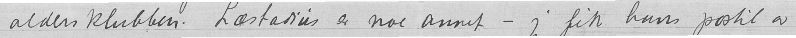aldersklubben. Læstadius er noe annet – jeg fik hans postil av
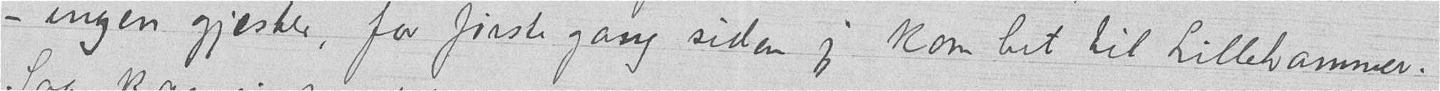– ingen gjester, for første gang siden jeg kom hit til Lillehammer.
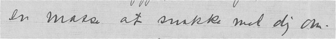en masse at snakke med dig om.
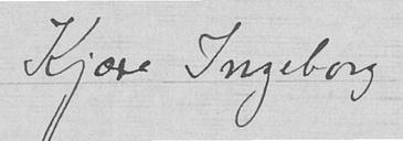Kjære Ingeborg
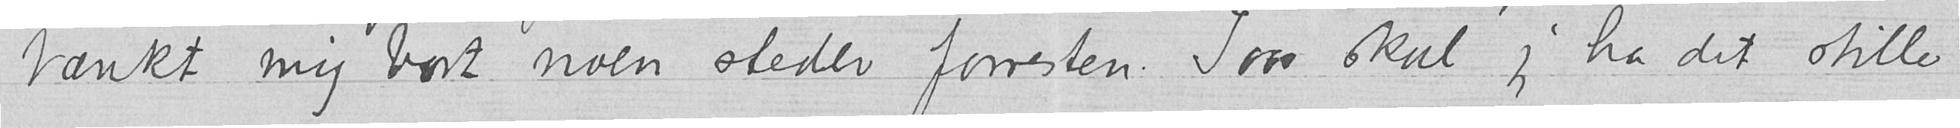tænkt mig bort noen steder forresten. I aar skal jeg ha det stille
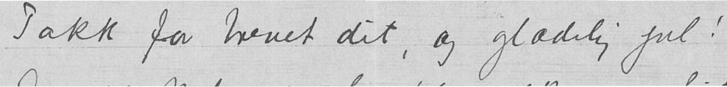Takk for brevet dit, og glædelig jul!
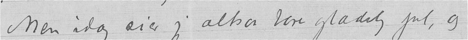Men idag sier jeg altsaa bare glædelig jul, og
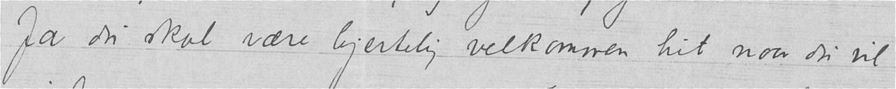Ja du skal være hjertelig velkommen hit naar du vil
